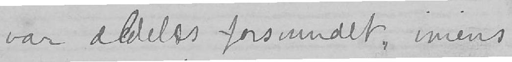var aldeles forsvundet, imens
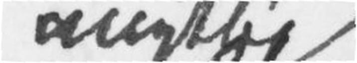unyttig
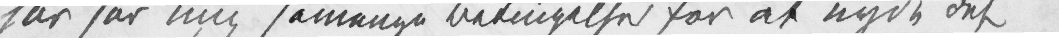har for mig såmange Betingelser for at nyde det
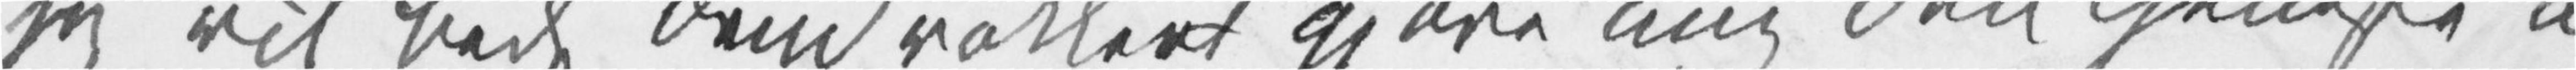jeg vil bede Dem vakkert gjøre mig den Tjeneste a[t]
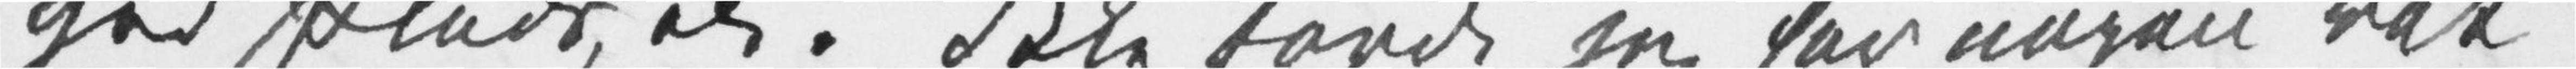god Plads, etc. Ikke fordi jeg har nogen ret
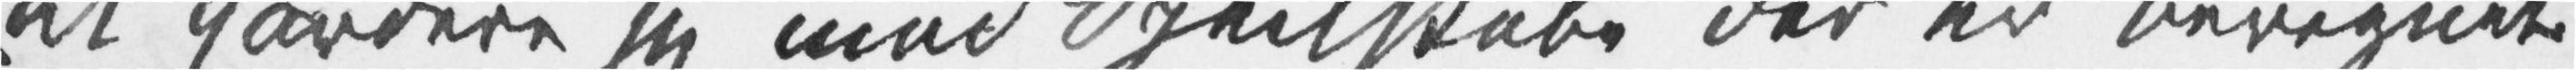at gardere sig mod Speilskabe der er beregnet
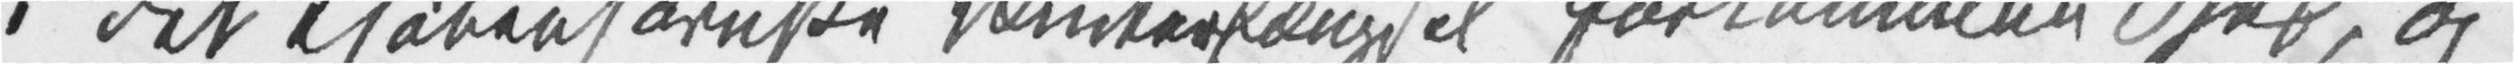i det Kjøbenhavnske Vinterfængsel forkommen Sjel, og
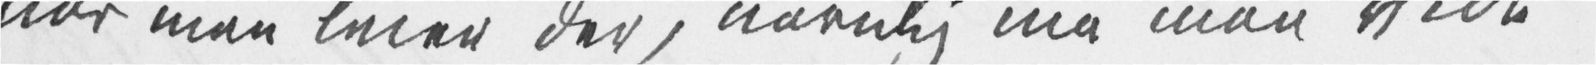når man leier der, navnlig må man vide
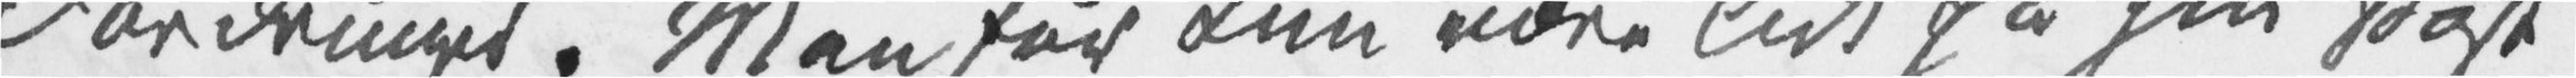Fordringer. Man får kun være lidt på sin Post
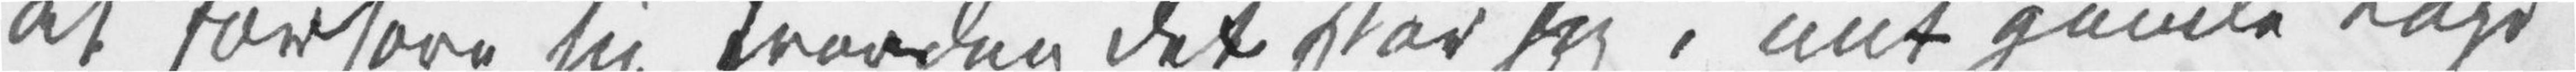at forhøre sig hvordan det står sig i mit gamle Logi
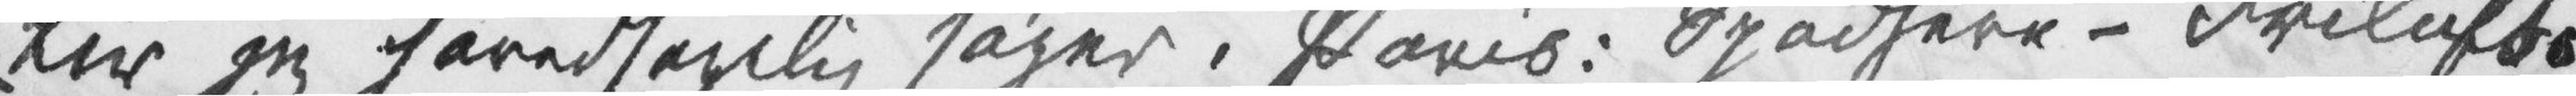Liv jeg hovedsagelig søger i Paris: Spadsere – Frilufts¬
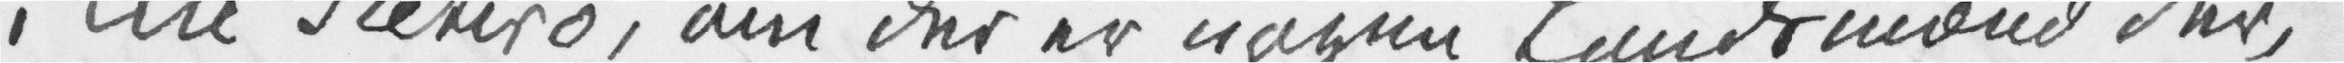i Cité Retiro, om der er nogen Landsmænd der,
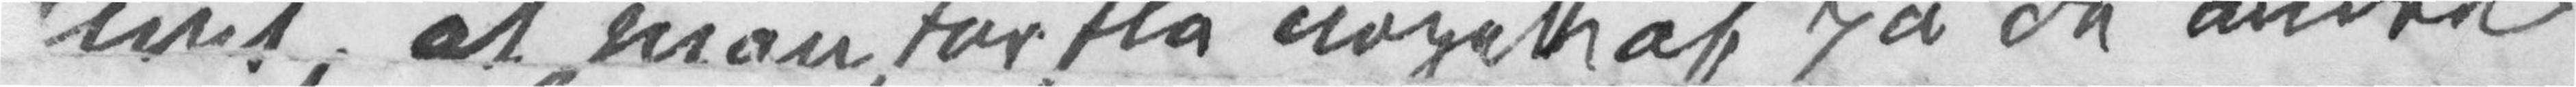livet, at man får slå noget af på de andre
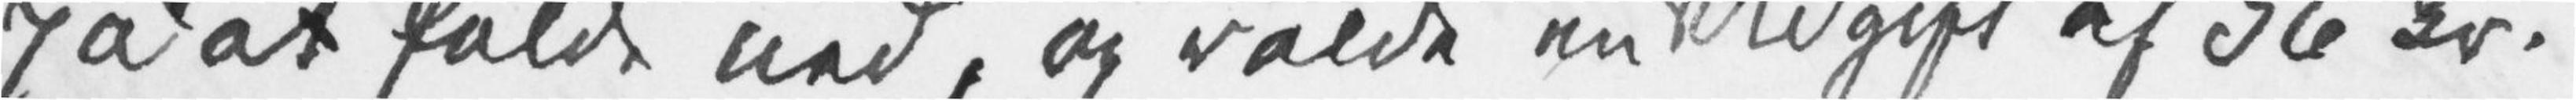på at falde ned, og volde en Udgift af 56 Kr.
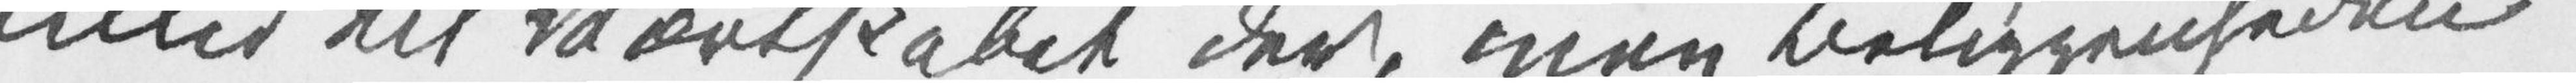Tillid til Værtskabet der, men Beliggenheden
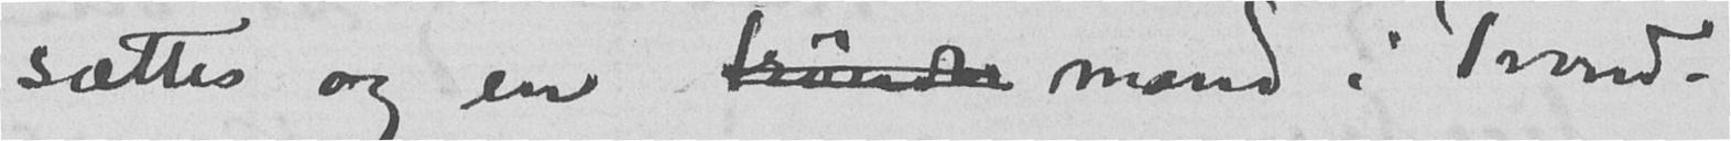sættes og en trønder mand i Trond-
